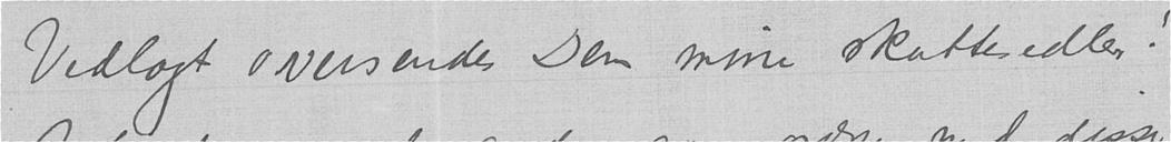Vedlagt oversendes Dem mine skattesedler!
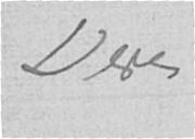Deres
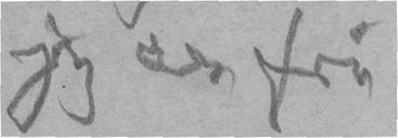jeg er fri
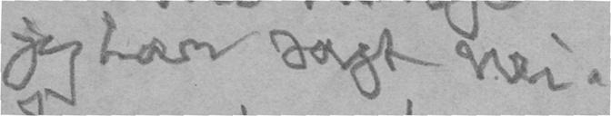jeg har sagt nei.
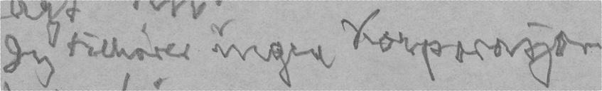Jeg tilhører ingen Korporasjon
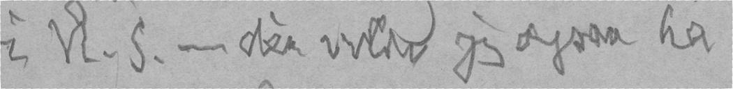i N. S. - dèr vilde jeg ogsaa ha
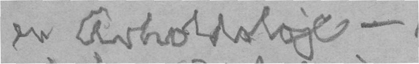en Avholdsloje -
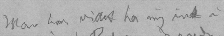Man har villet ha mig ind i
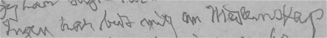Ingen har bedt mig om Medlemskap
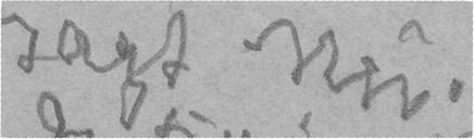sagt nei.
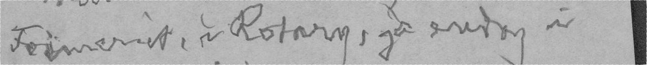Frimeriet, i Rotary, ja endog i
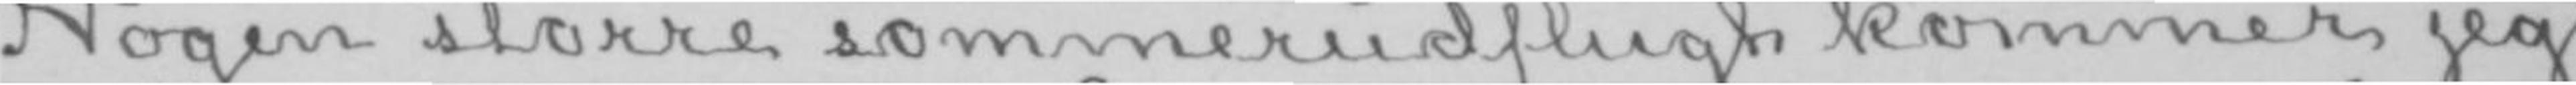Nogen større sommerudflugt kommer jeg
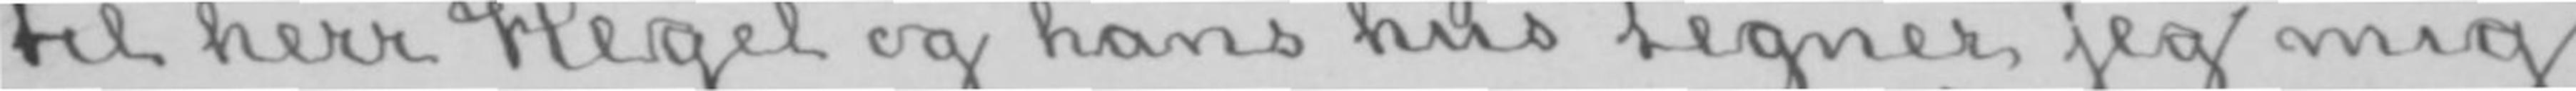til herr Hegel og hans hus tegner jeg mig
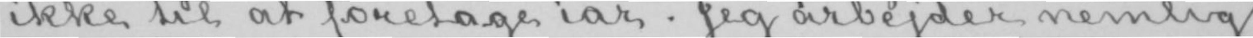ikke til at foretage iår. Jeg arbejder nemlig
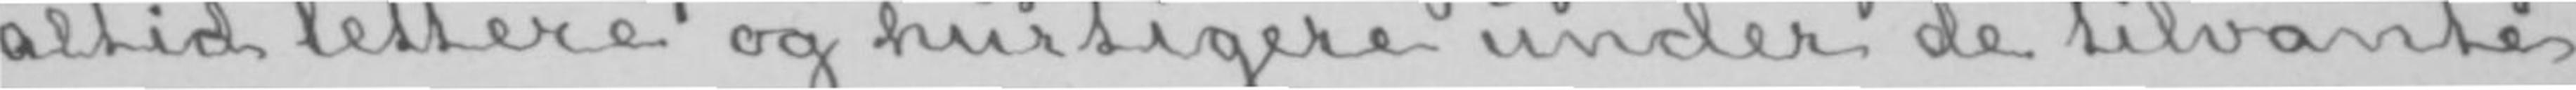altid lettere og hurtigere under de tilvante
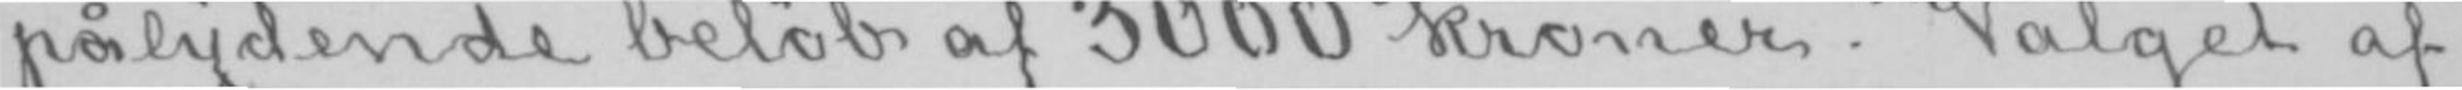pålydende beløb af 3000 kroner. Valget af
